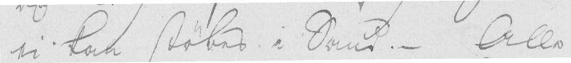vi kan støbes i Sand. - Alle
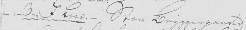"- "-3-" I Leer. - Store Bryggerpander,
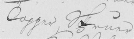Tapper, Skruver
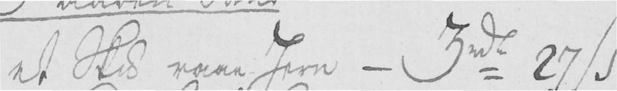et Skpd raae Jern - 3 rde 27 s
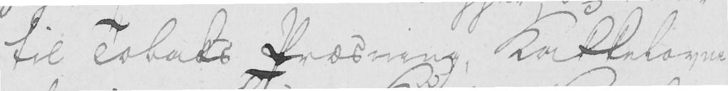til Tobaks Præsning, Kakkelovne
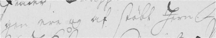gen ere og af støbt Jern. -
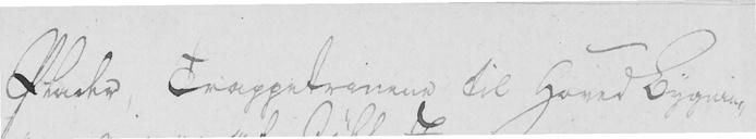Plader, Trappetrinner til Hoved Bygnin-
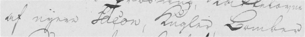af nyere Facon, Kugler, Bomber
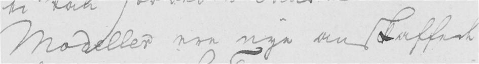Modeller ere nye anskaffede
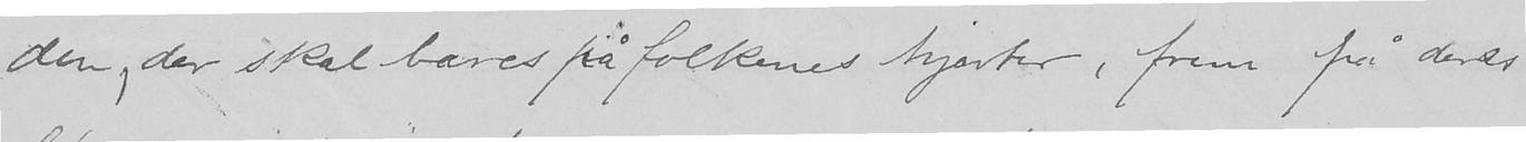den, der skal bæres på folkenes hjærter, frem på deres
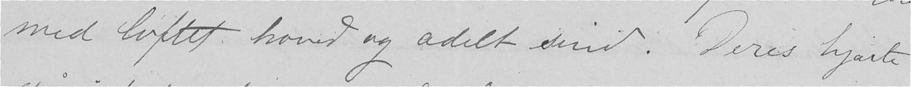med løftet hoved og ædelt sind. Deres hjærte
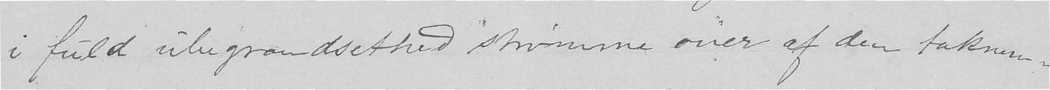i fuld ubegrændsethed strømme over af den taknem-
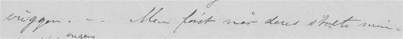vuggen. - - Men først når deres stolte min-
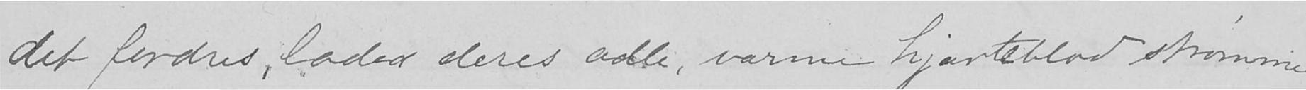det fordres lader deres ædle, varme hjærteblod strømme
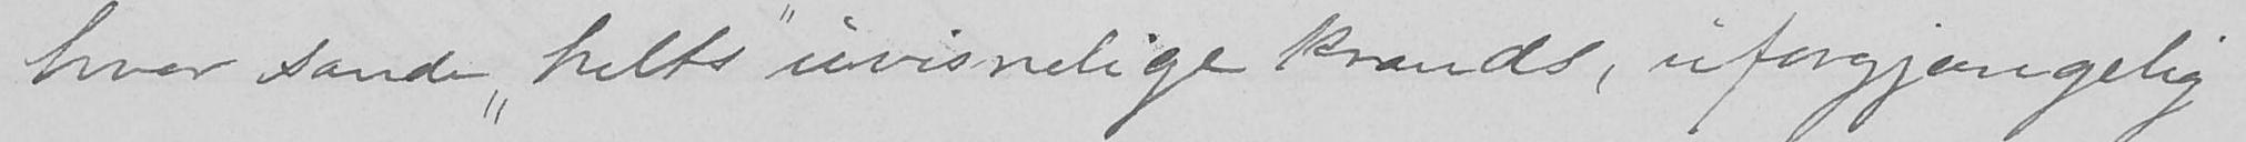hver sande "helts" uvisnelige krands, uforgjengelig
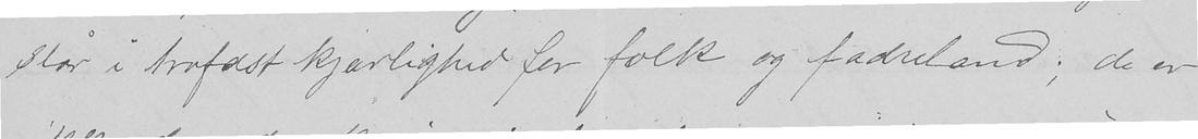slår i trofast kjærlighed for folk og fædreland; de er
